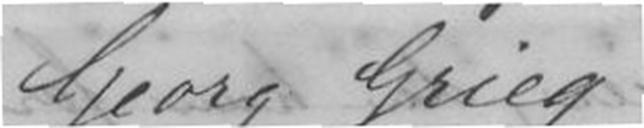Georg Grieg
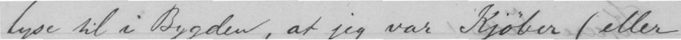lyse til i Bygden, at jeg var Kjøber (eller
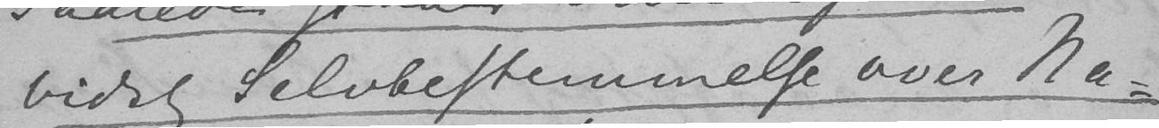vidst Selvbestemmelse over Na-
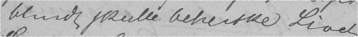blindt skulle beherske Livet.
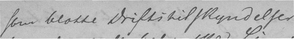som blotte Driftstilskyndelser
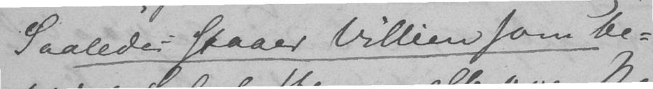Saaledes staaer Villien som be-
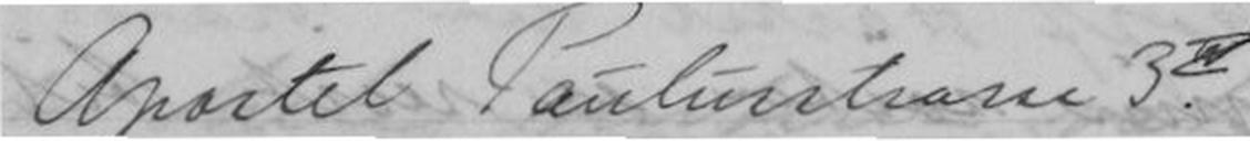Apostel Paulusstrasse 3.IV
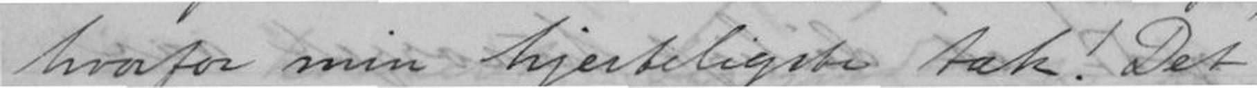hvorfor min hjerteligste tak! Det
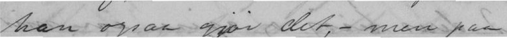han ogsaa gjør det, - men paa
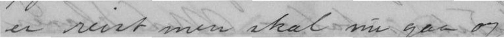er reist men skal nu gaa og
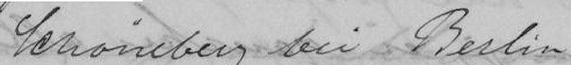Schöneberg bei Berlin
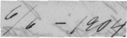6/6-1904
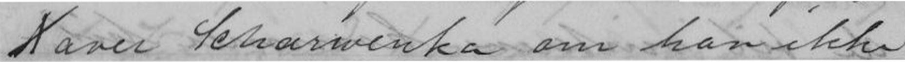Xaver Scharwenka om han ikke
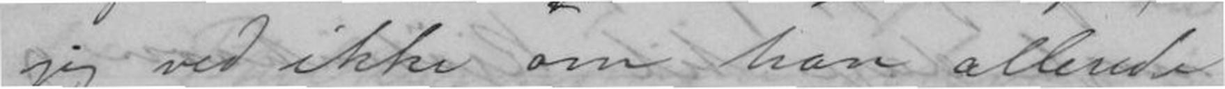jeg ved ikke om han allerede
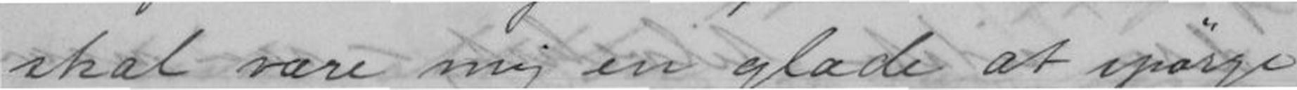skal være mig en glæde at spørge
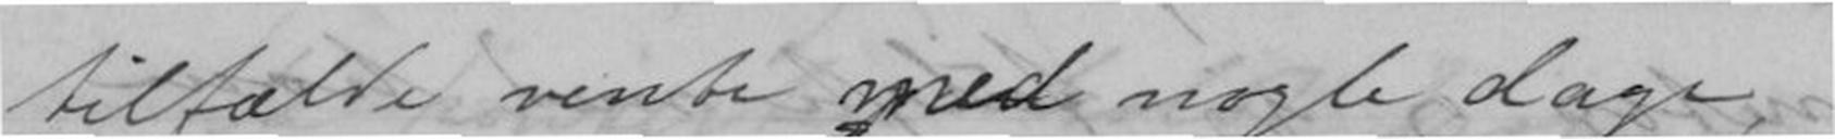tilfælde vente med nogle dage,
